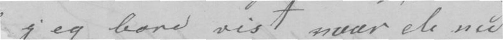jeg bare vist naar de nu
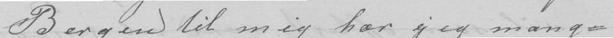Bergen til mig har jeg mang-
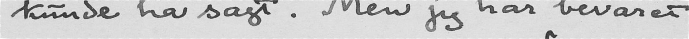kunde ha sagt! Men jeg har bevaret
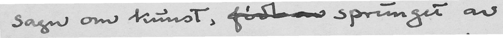sagn om kunst, gid hen sprunget av
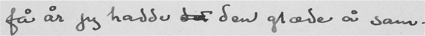få år jeg hadde det den glæde å sam-
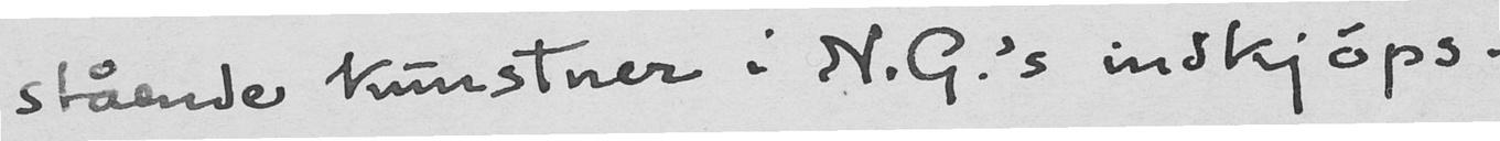stående kunstner i N.G.'s indkjøps-
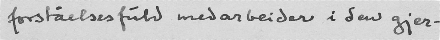forståelsesfuld medarbeider i den gjer-
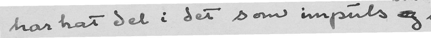har hat del i det som impuls og
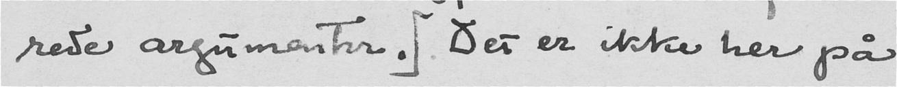rede argumenter. Det er ikke her på
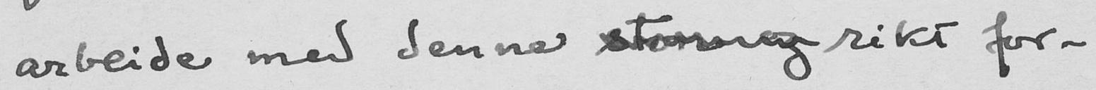arbeide med denne stormig rikt for-
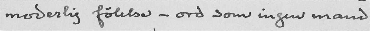moderlig følelse - ord som ingen mand
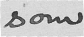som
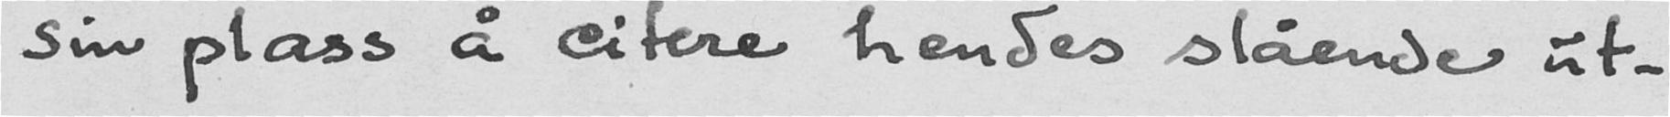sin plass å citere hendes slående ut-
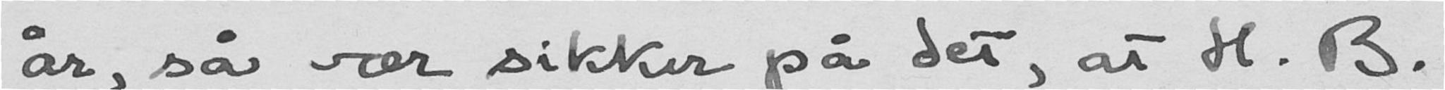år, så vær sikker på det, at H. B.
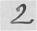2
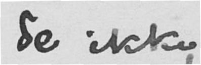de ikke
